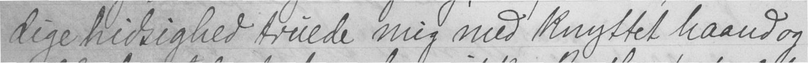dige hidsighed truede mig med knyttet haand og
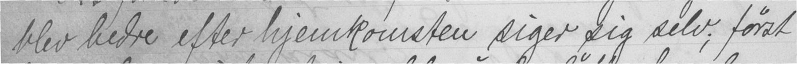blev bedre efter hjemkomsten siger sig selv; først
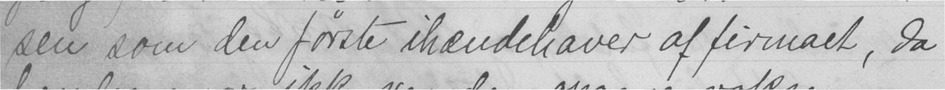sen som den første ihændehaver af firmaet, da
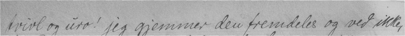tvivl og uro! jeg gjemmer den fremdeles og ved ikke,
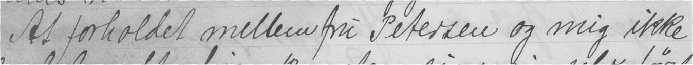At forholdet mellem fru Petersen og mig ikke
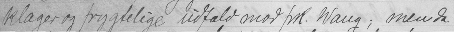klager og frygtelige udfald mod frk. Wang; men da
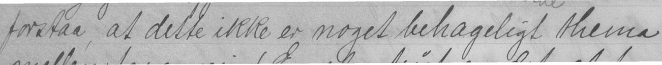forstaa, at dette ikke er noget behageligt thema
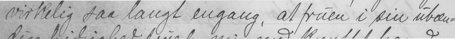virkelig saa langt engang, at fruen i sin ubæn-
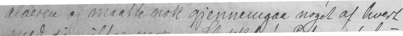alderen og maatte nok gjennemgaa noget af hvert
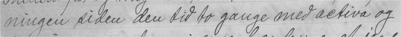ningen siden den tid to gange med activa og
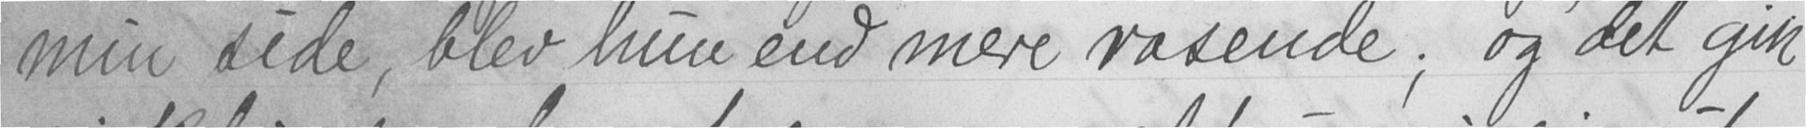min side, blev hun end mere rasende; og det gik
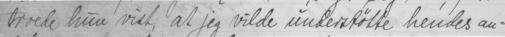troede hun vist, at jeg vilde understøtte hendes an-
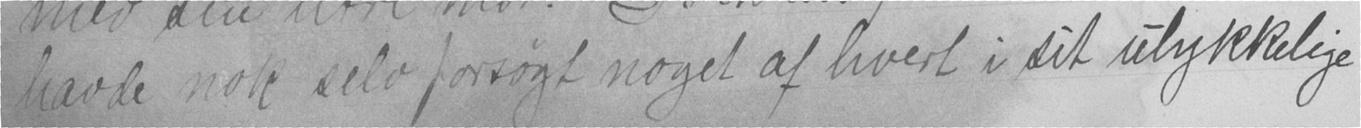havde nok selv forsøgt noget af hvert i sit ulykkelige
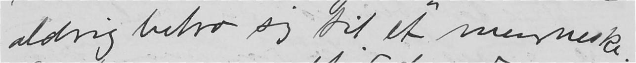aldrig betro sig til et menneske.
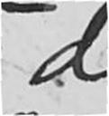de
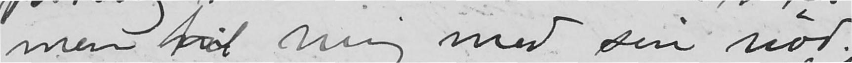men vil mig med sin nød.
